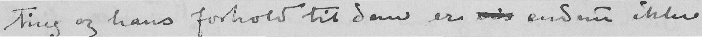ting og hans forhold til dem er vis endnu ikke
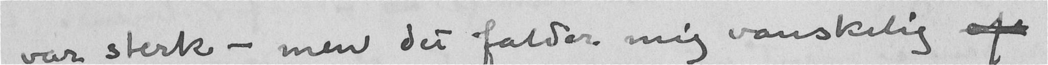var sterk - men det falder mig vanskelig ef
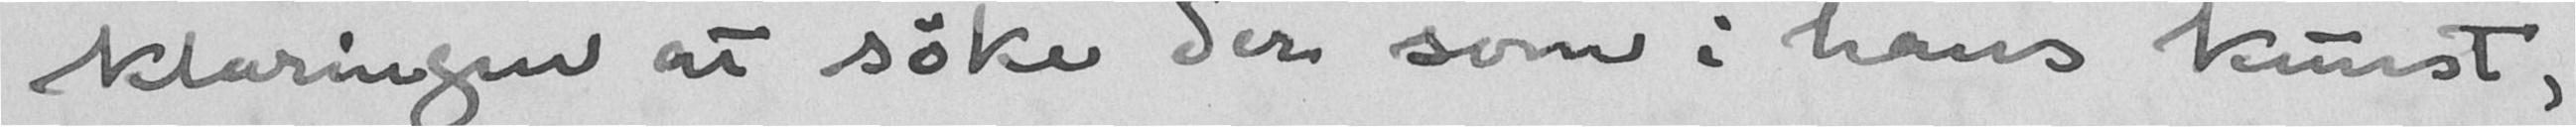klaringen at søke der som i hans kunst,
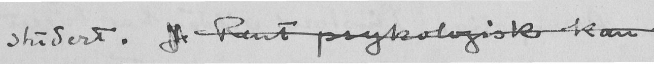studert. Rent psykologisk kan
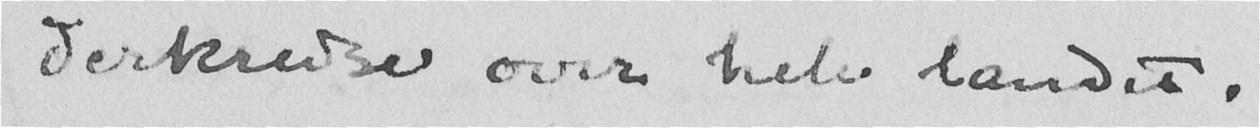derkredse over hele landet.
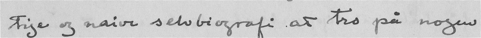tige og naive selvbiografi at tro på nogen
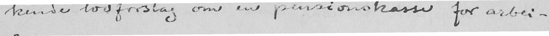kende lovforslag om en pensionskasse for arbei-
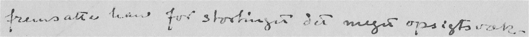fremsatte han for stortinget det meget opsigtsvæk-
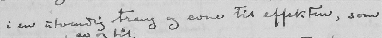i en utvendig trang og evne til effekten, som
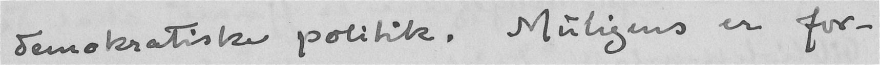demokratiske politik. Muligens er for-
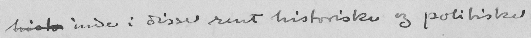histo inde i disse rent historiske og politiske
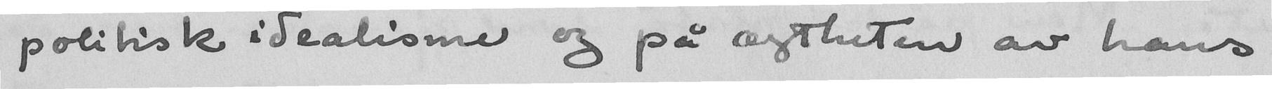politisk idealisme og på ægtheten av hans
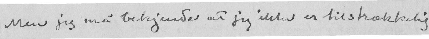Men jeg må bekjende at jeg ikke er tilstrækkelig
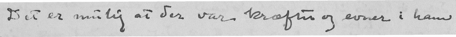Det er mulig at der var kræfter og evner i ham
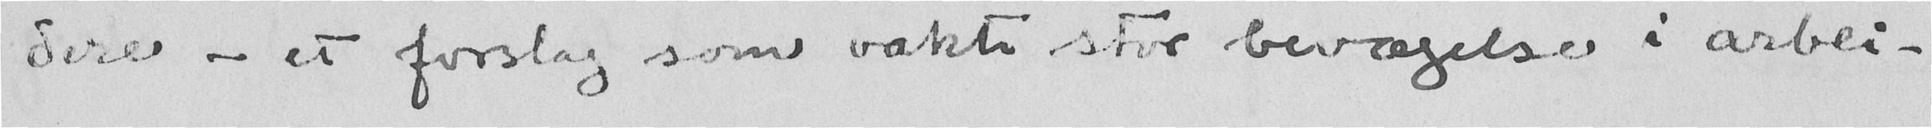dere - et forslag som vakte stor bevægelse i arbei-
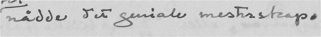nådde det geniale mesterskap.
